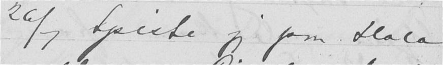26/7 Spiste jeg paa Hola
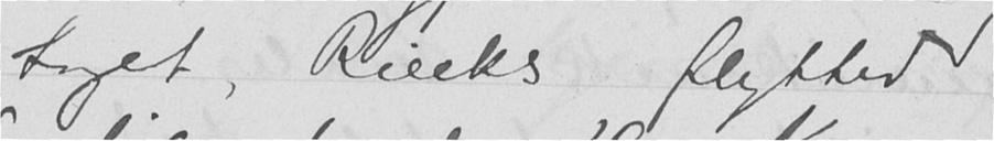toget, Riecks flytted
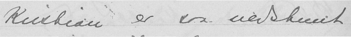Kristian er saa nedstemt
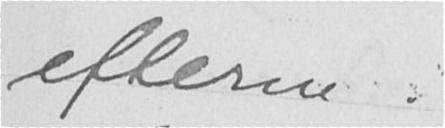efterm.
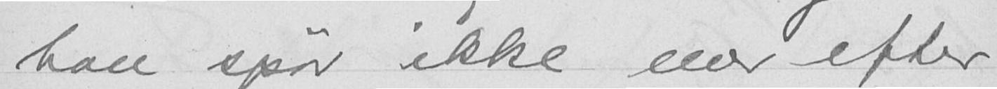han spør ikke mer efter
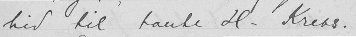hid til tante H. Krebs.
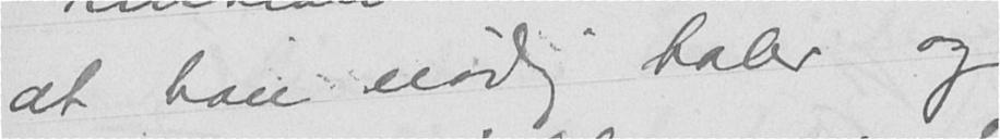at han nødig taler og
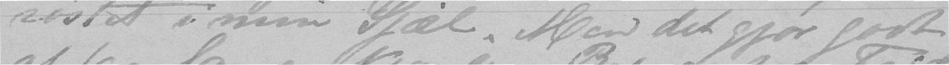røstet i min Sjæl. Men det gjør godt
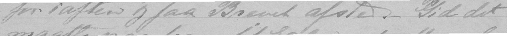for iaften og faa Brevet afsted. Gid det
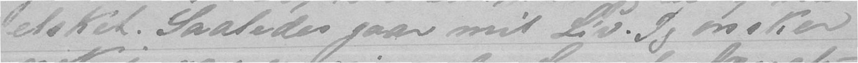elsket. Saaledes gaar mit Liv. Jeg ønsker
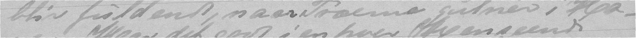blir fuldendt, naar Trærne gulner i Ha-
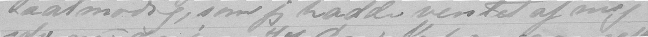Taalmodig, som jeg hadde ventet af mig
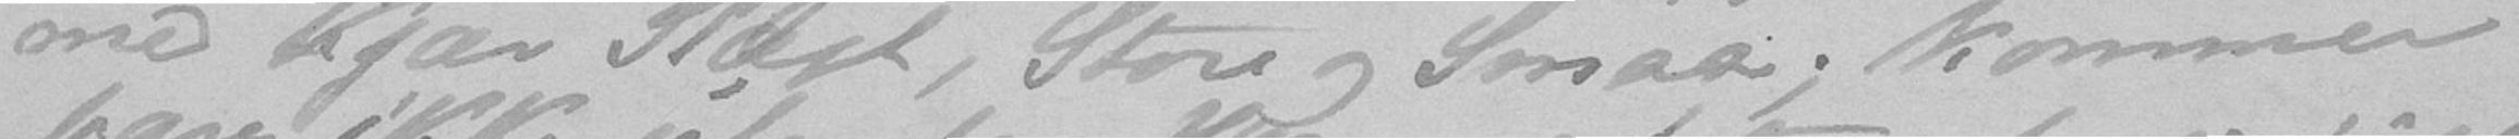med kjær Slægt, Store og Smaa; kommer
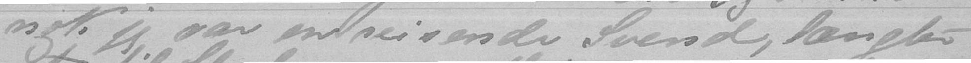nok jeg var en reisende Svend, længter
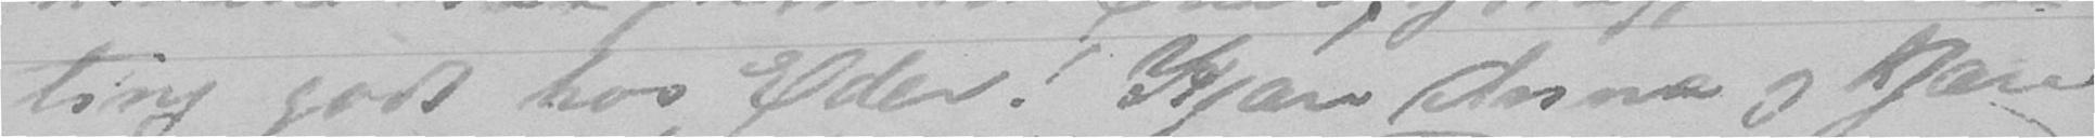ting godt hos Eder. Kjære Anna og kjære
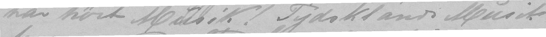har hørt Musik! Tydsklands Musik
